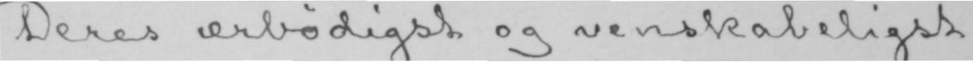Deres ærbødigst og venskabeligst
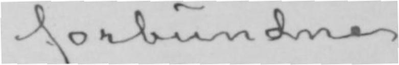forbundne
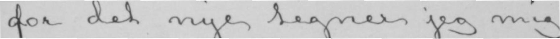for det nye tegner jeg mig
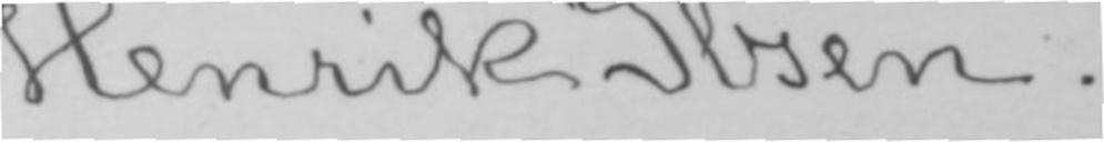Henrik Ibsen.
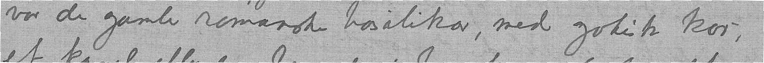var de gamle romanske basilikaer med gotisk kor,
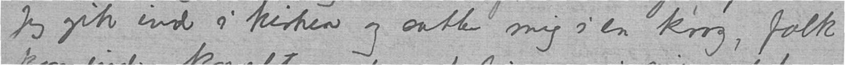Jeg gik ind i kirken og satte mig i en krog, folk
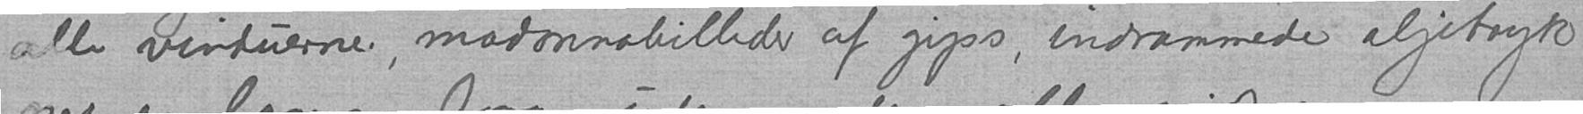alle vinduerne, madonnabilleder af gips, indrammede oljetryk
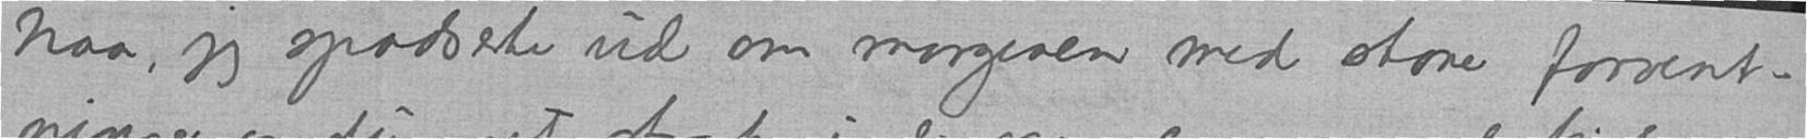Naa, jeg spadserte ud om morgenen med store forvent-
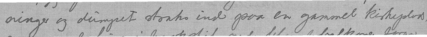ninger og dumpet straks ind paa en gammel kirkeplads,
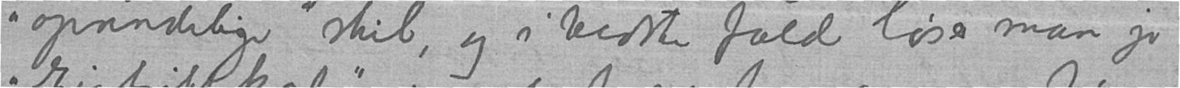"oprindelige" stil, og i bedste fald løser man jo
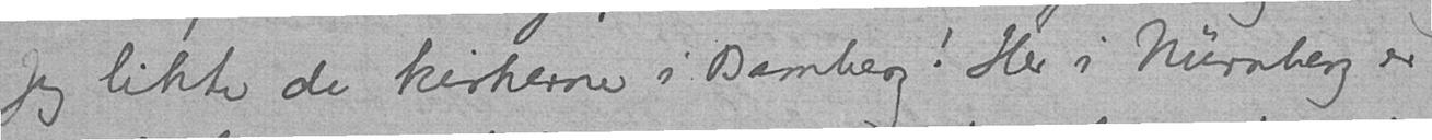Jeg likte de kirkerne i Bamberg! Her i Nürnberg er
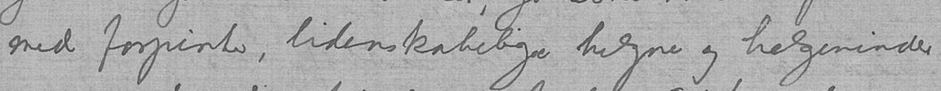med forpinte, lidenskabelige helgner og helgeninder
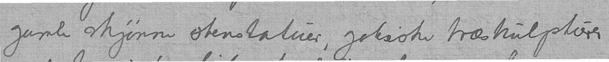gamle skjønne stenstatuer, gotiske træskulpturer
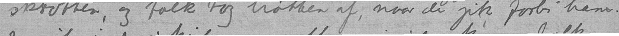skrotten, og folk tog hatten af, naar de gik forbi ham.
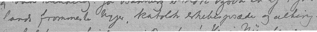lands frommeste byer, katolsk erkebispesæde og alting.
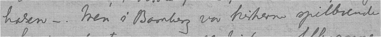halsen -. Men i Barnberg var kirkerne spillevende
